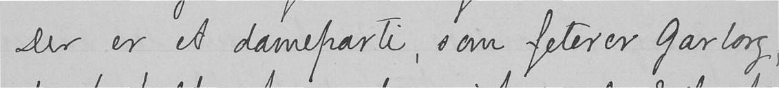Der er et dameparti, som feterer Garborg,
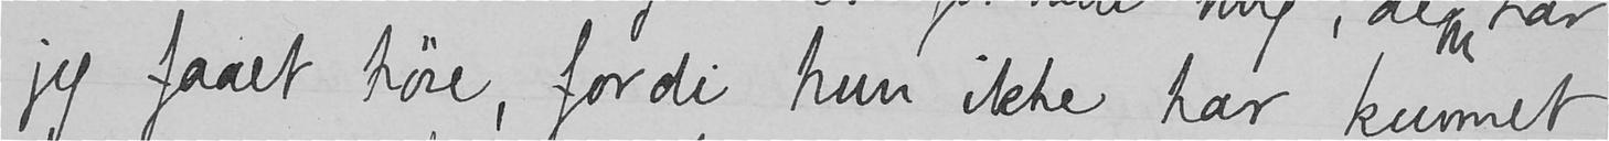jeg faaet høre, fordi hun ikke har kunnet
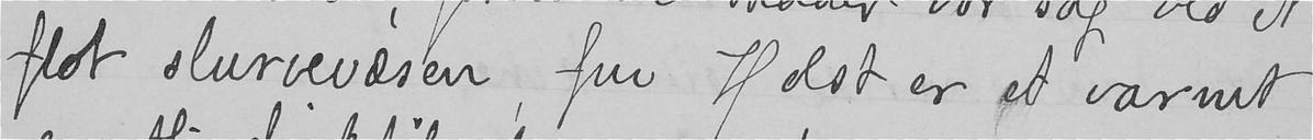flot slurvevæsen, fru Holst er et varmt
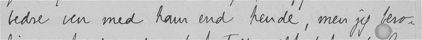bedre ven med ham end hende, men jeg bero-
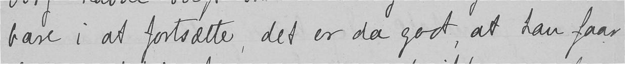bare i at fortsætte, det er da godt, at han faar
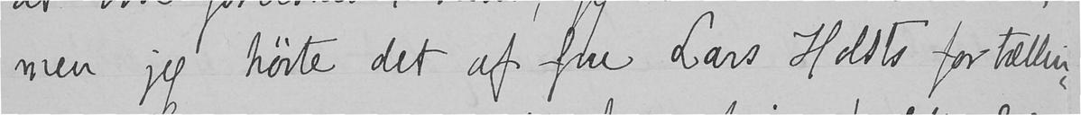men jeg hørte det af fru Lars Holsts fortællin-
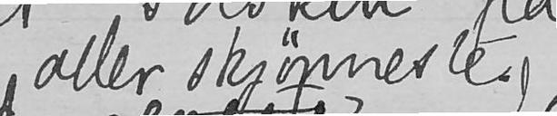aller skjønneste.
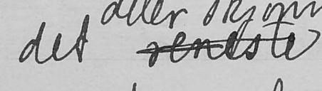det reneste
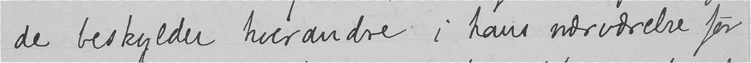de beskylder hverandre i hans nærværelse for
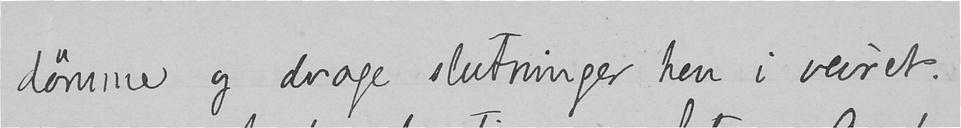dømme og drage slutninger hen i veiret.
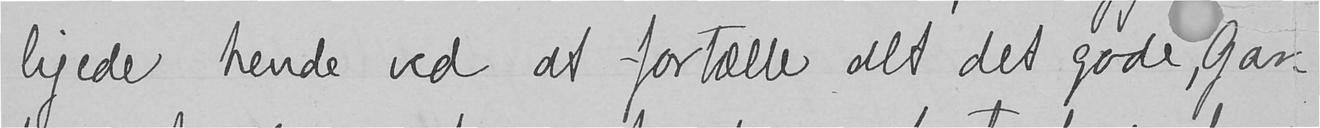ligede hende ved at fortælle alt det gode, Gar-
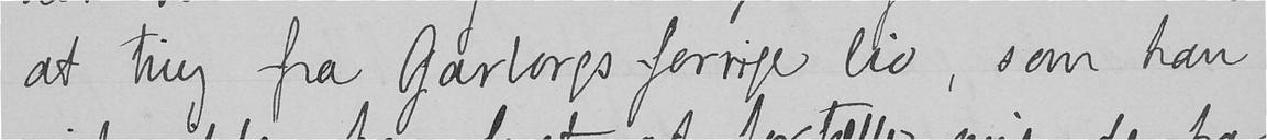at ting fra Garborgs forrige liv, som han
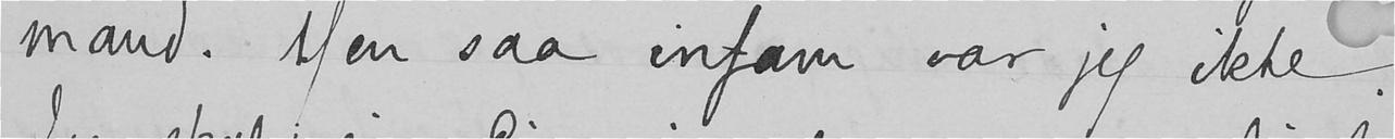mand. Men saa infam var jeg ikke.
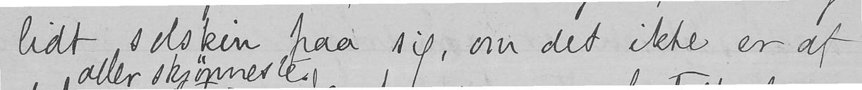lidt solskin paa sig, om det ikke er af
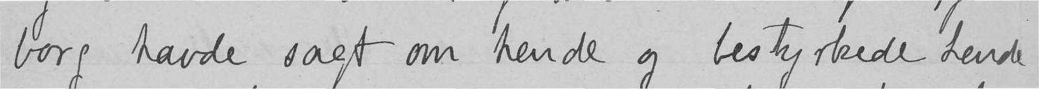borg havde sagt om hende og bestyrkede hende
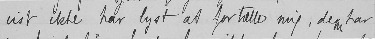vist ikke har lyst at fortælle mig, dem har
